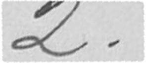2.
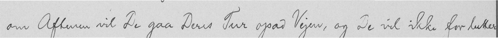om Aftenen vil De gaa Deres Tur opad Vejen, og De vil ikke for lutter
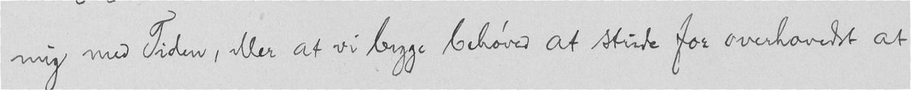mig med Tiden, eller at vi begge behøver at stride for overhovedet at
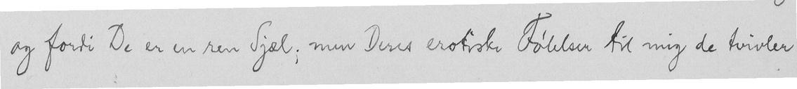og fordi De er en ren Sjæl; men Deres erotiske Følelser til mig de tvivler
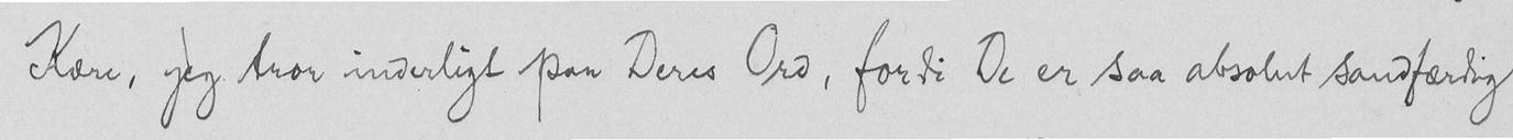Kære, jeg tror inderligt paa Deres Ord, fordi De er saa absolut sandfærdig
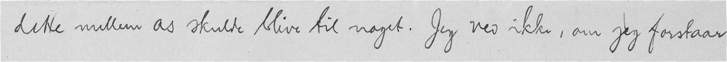dette mellem os skulde blive til noget. Jeg ved ikke, om jeg forstaar
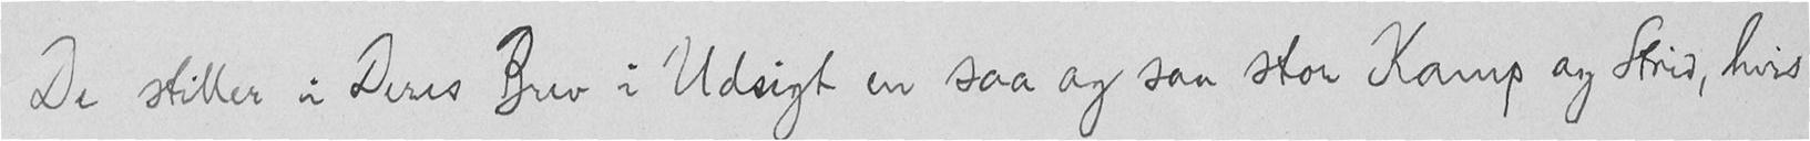De stiller i Deres Brev i Udsigt en saa og saa stor Kamp og Strid, hvis
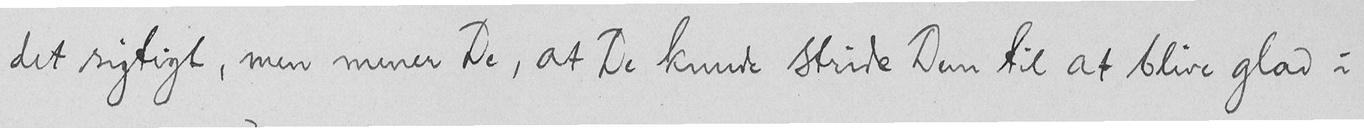det rigtigt, men mener De, at De kunde stride Dem til at blive glad i
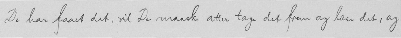De har faaet det, vil De maaske atter tage det frem og læse det, og
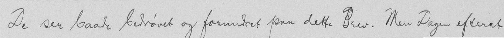De ser baade bedrøvet og forundret paa dette Brev. Men Dagen efterat
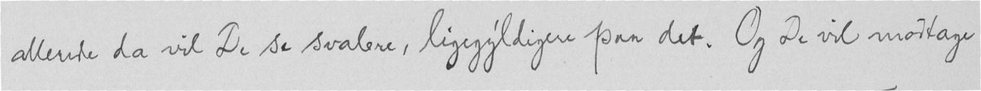allerede da vil De se svalere, ligegyldigere paa det. Og De vil modtage
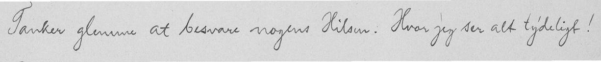Tanker glemme at besvare nogens Hilsen. Hvor jeg ser alt tydeligt!
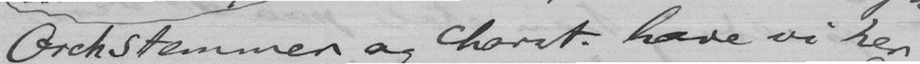Orchstemmer og Choret. have vi her
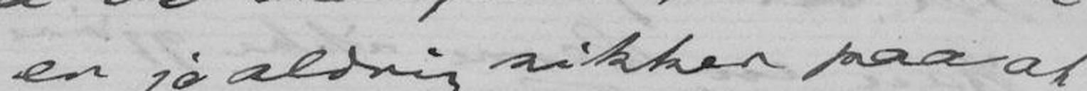er jo aldrig sikker paa at
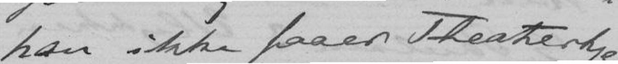han ikke faaer Theatertjeneste.
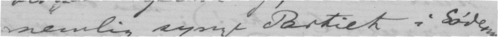nemlig synge Partiet i Sødermanns
